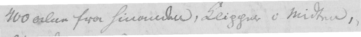400 alen fra hinanden, Klipper i Midten,
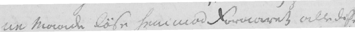ne Maade løse henimod Foraaret alle disse
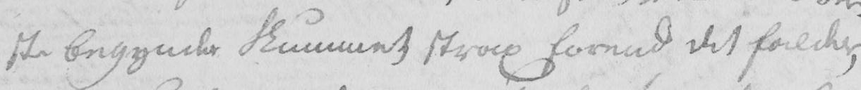ste begynder Skummet strax førend det falder,
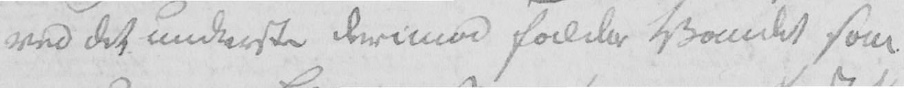ved det underste derimod falder Vandet som
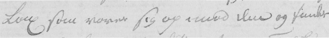Lax som vover sig op imod den og finder
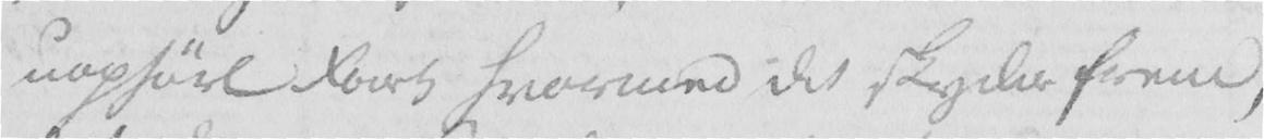uophørl Fart hvormed det skyder frem,
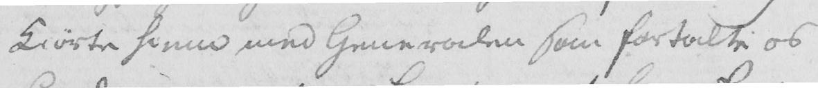Kiørte hiem med Generalen som fortalte os
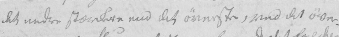det nedre stærkere end det øverste, ved det øver-
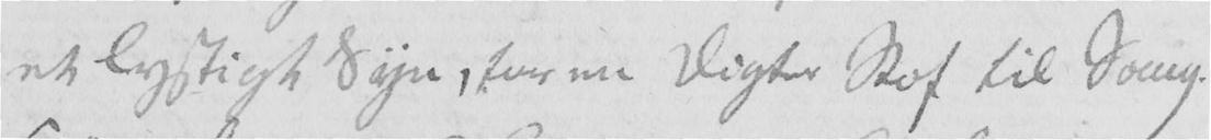et lystigt Syn, for en Digter Stof til Sang.
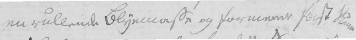en rullende Blyemasse og formerer først Skum
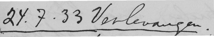24.7.33 Veslevangen.
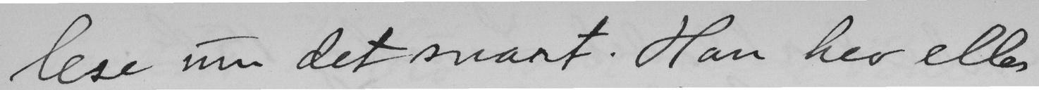lese um det snart. Han hev elles
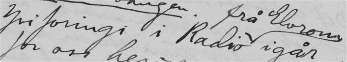Yviforingi i Radio frå Elverom i går
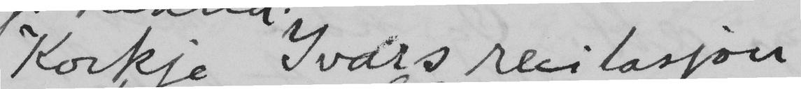Korkje Ivars recitasjon
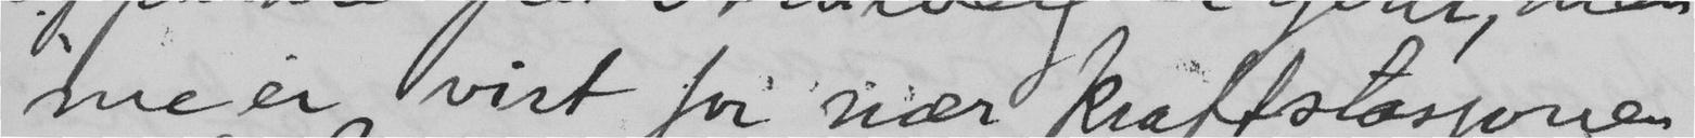me er vist for nær kraftstasjonen
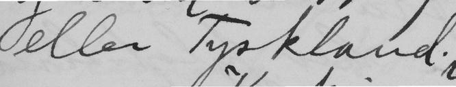eller Tyskland.
